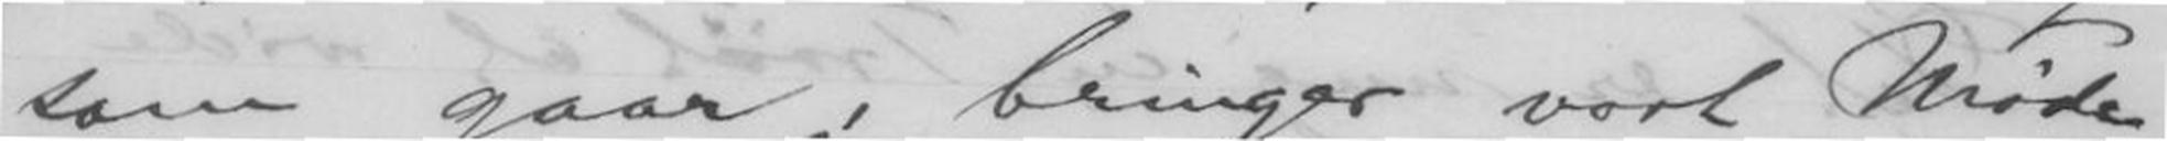som gaar, bringer vort Møde
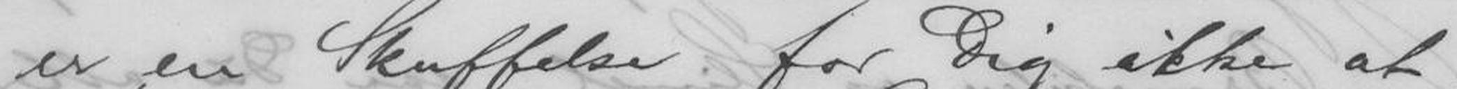er en Skuffelse for Dig ikke at
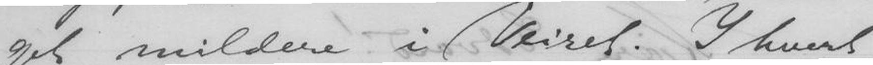get mildere i Veiret. I hvert
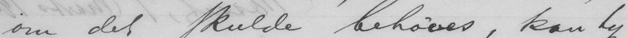om det skulde behøves, kan ty
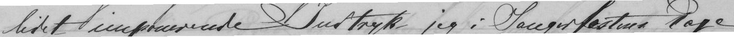lidet imponerende Indtryk jeg i Sangerfestens Dage
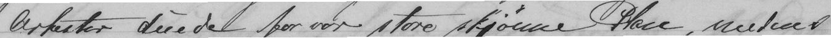Orkester duede for vor store skjønne Plan, medens
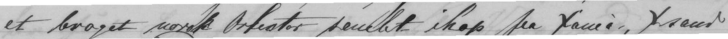et broget norsk Orkester samlet ihop fra Xania, Xsand,
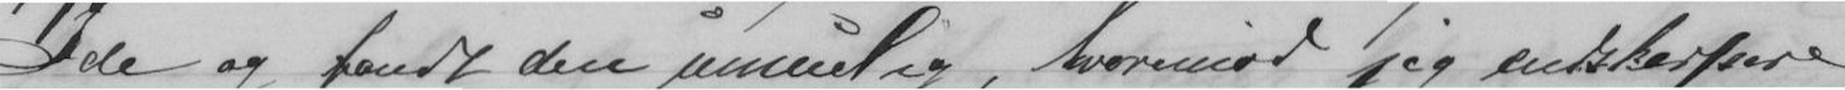Ide og fandt den umuelig, hvorimod jeg endskarpere
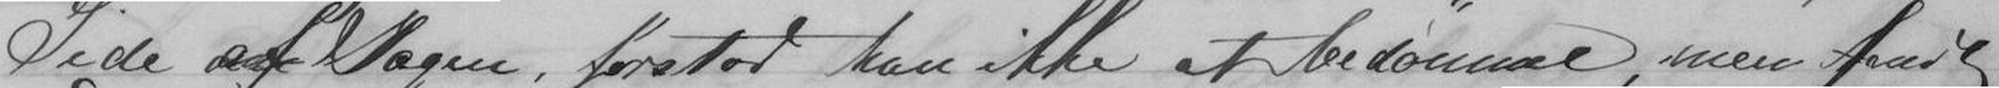Side af Sagen, forstod han ikke at bedømme, men fandt
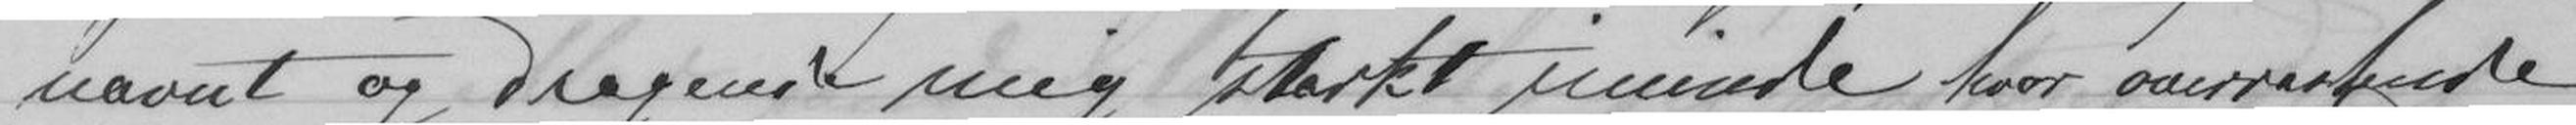nævnt og dragende mig stærkt iminde hvor overraskende
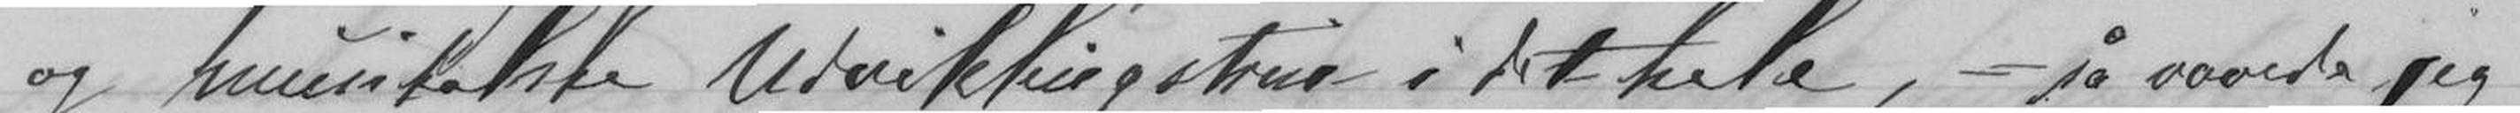og musikalske Udviklingstrin i det hele, så vovede jeg
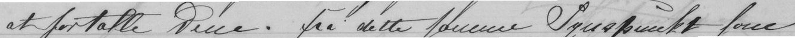at fortælle Dem. Fra dette samme Synspunkt som
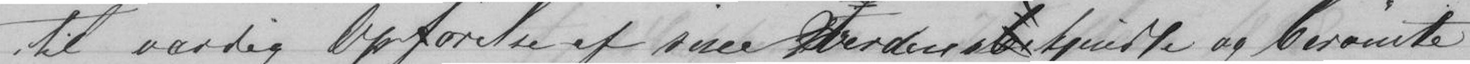til værdig Opførelse af sine verdensbkjendte og berømte
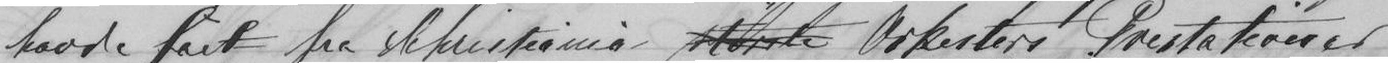havde fået fra Christianias største Orkesters Prestationer
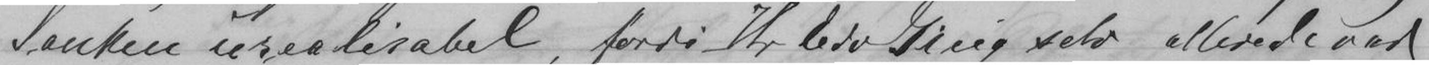Tanken urealisabel, fordi Hr Edv. Grieg selv allerede var
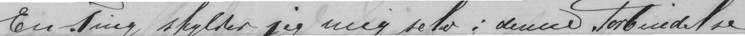En Ting skylder jeg mig selv i denne Forbindelse
